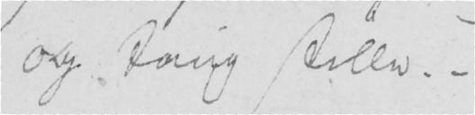og taug stille. -
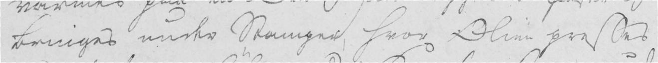bringes under Stamper, hvor Olien presses
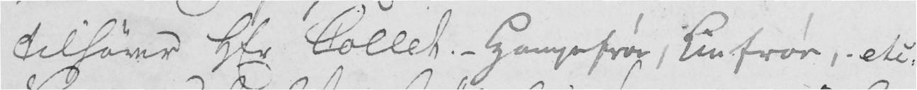tilhører Hr Collet. - Hampefrøe, Linfrøe, - etc.
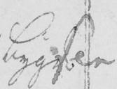bygsler
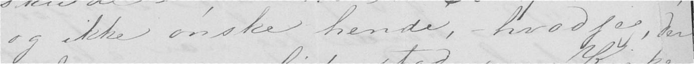og ikke ønske hende, - hvadfor, der
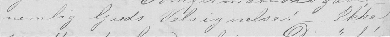nemlig Guds Velsignelse! - - Ikke
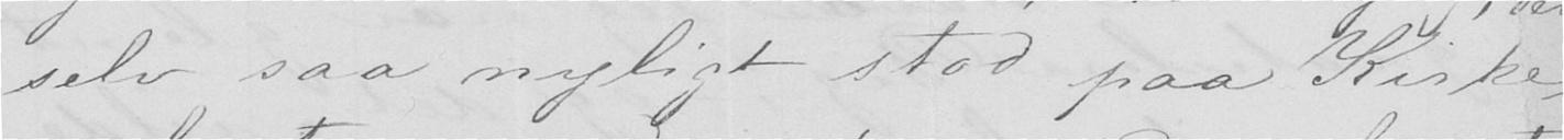selv saa nyligt stod paa Kirke-
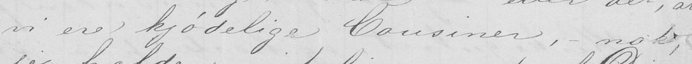vi ere kjødelige Cousiner, - nok,
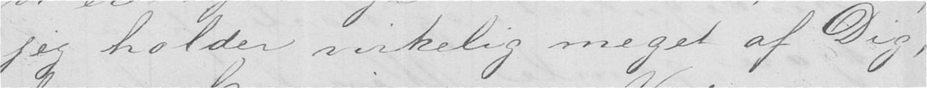jeg holder virkelig meget af Dig,
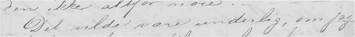- Det vilde være underlig om jeg
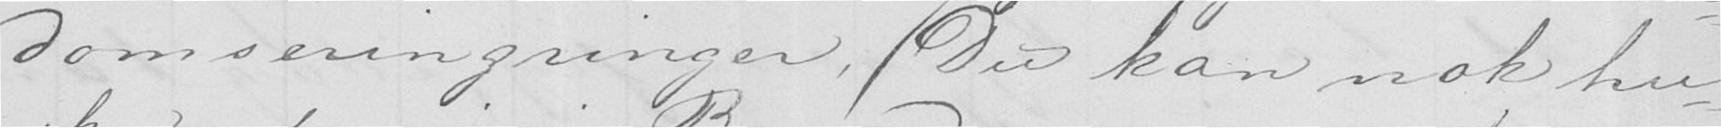domseringringer, (Du kan nok hu-
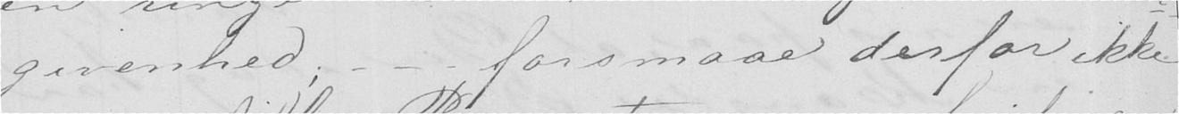givenhed; - - - forsmaae derfor ikke
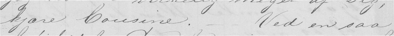kjære Cousine. - - Ved en saa
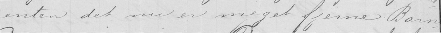enten det nu er meget fjerne Barn-
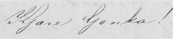Kjære Gonka!
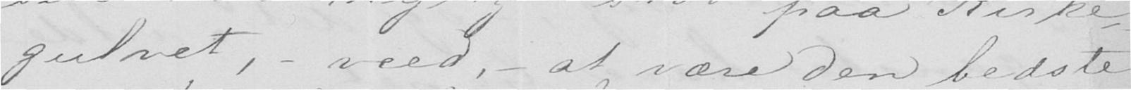gulvet, - veed, - at være den bedste
